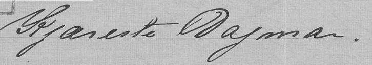Kjæreste Dagmar.
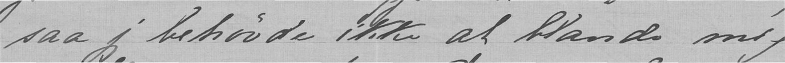saa jeg behøvde ikke at blande mig
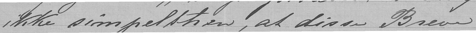ikke simpelthen, at disse Breve
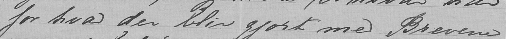for hvad der blir gjort med Brevene,
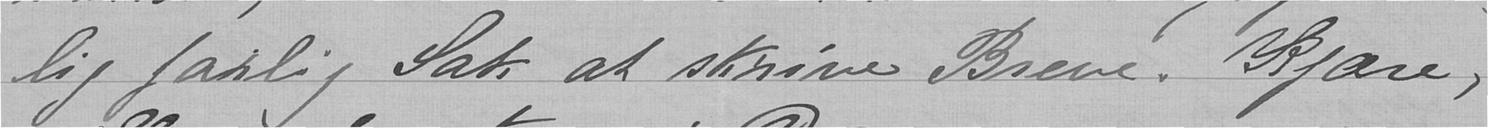lig farlig Sak at skrive Breve. Kjære,
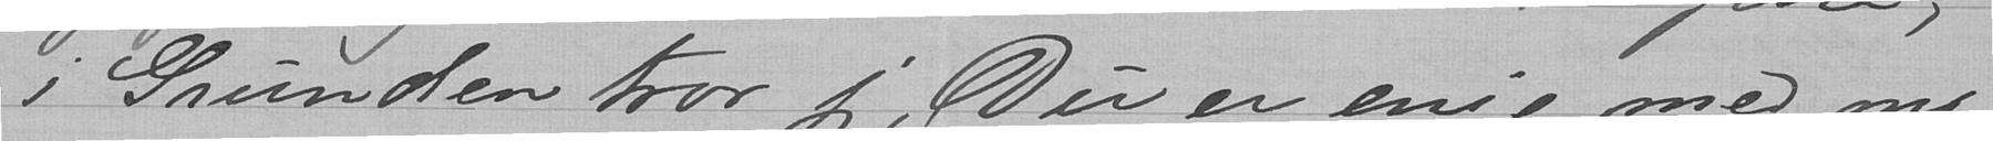i Grunden tror jeg, Du er enig med mig
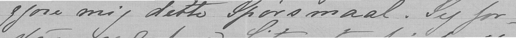gjøre mig dette Spørsmaal. Jeg for-
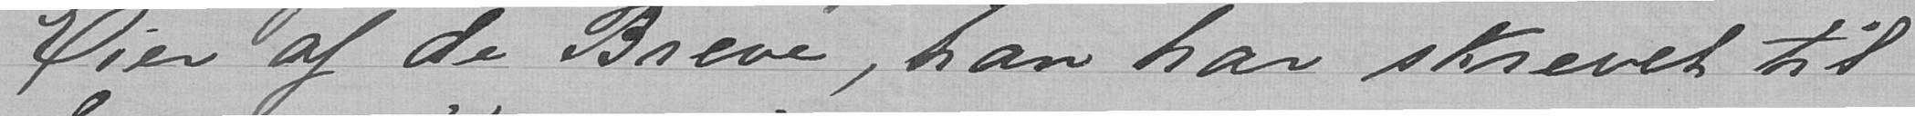Eier af de Breve, han har skrevet til
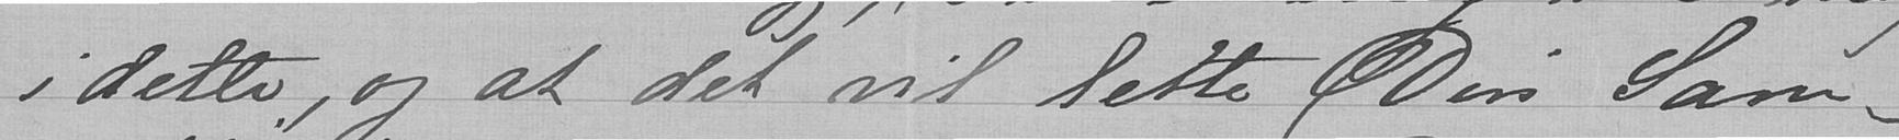i dette, og at det vil lette Din Sam-
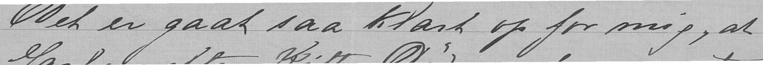Det er gaat saa klart op for mig, at
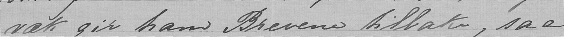væk gir ham Brevene tilbake, saa
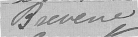Brevene
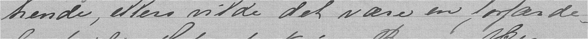hende, ellers vilde det være en forfærde-
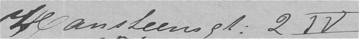Hansteensgt. 2 IV
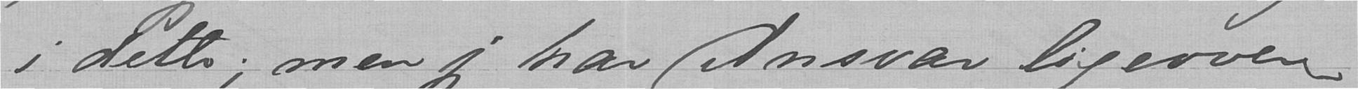i dette; men jeg har Ansvar ligeover-
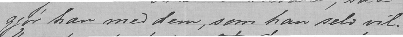gjør han med dem, som han selv vil.
